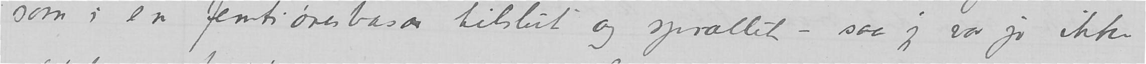som i en femtiøresbasar tilslut og sprællet – saa jeg var jo ikke
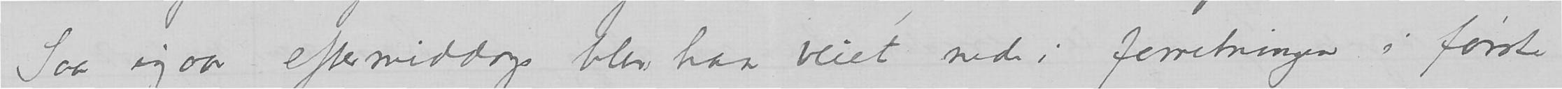Saa igaar eftermiddags blev han veiet nede i forretningen i første
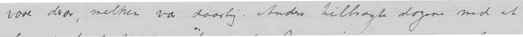være derav, melken var daarlig. Anders tilbragte dagene med at
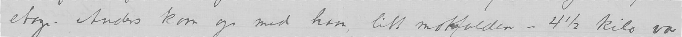etage. Anders kom op med ham, litt motfalden – 4 1/2 kilo var
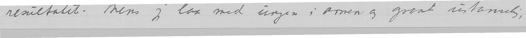resultatet. Mens jeg laa med ungen i armen og graat ustanselig,
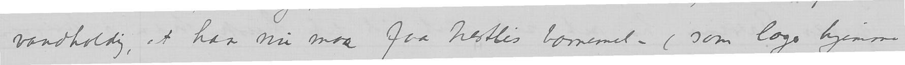vandholdig, at han nu maa faa Nestles barnemel – (som læger hjemme
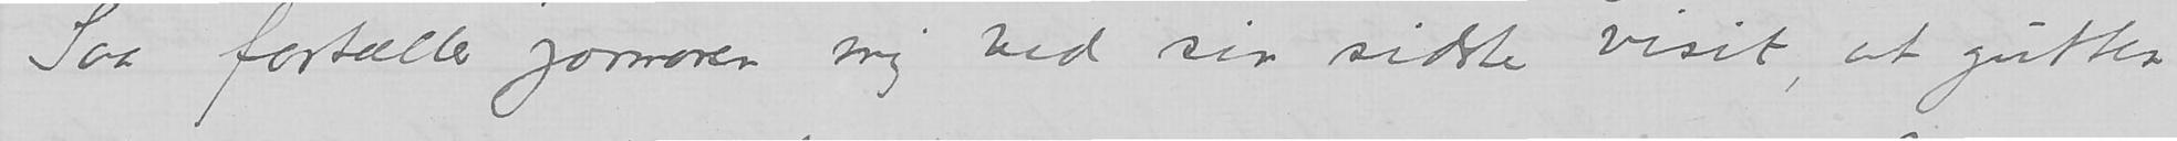–Så fortæller jormoren mig ved sin sidste visit, at gutten
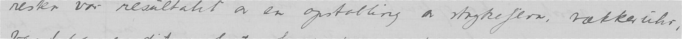resten var resultatet av en opstabling av strykejern, vækkeruhr,
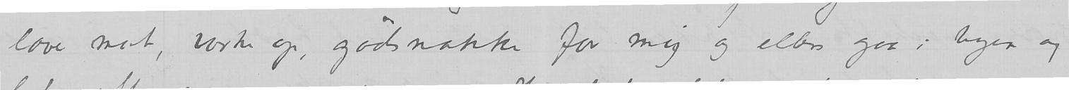lave mat, vaske op, godsnakke for mig og ellers gaa i byen og
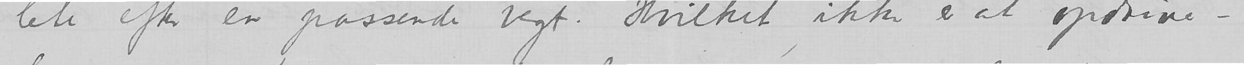lete efter en passende vegt. Hvilket ikke er at opdrive –.
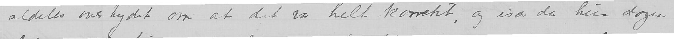aldeles overtydet om at det var helt korrekt, og især da hun dagen
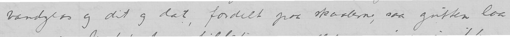vandglas og dit og dat, fordelt paa skaalerne, saa gutten laa
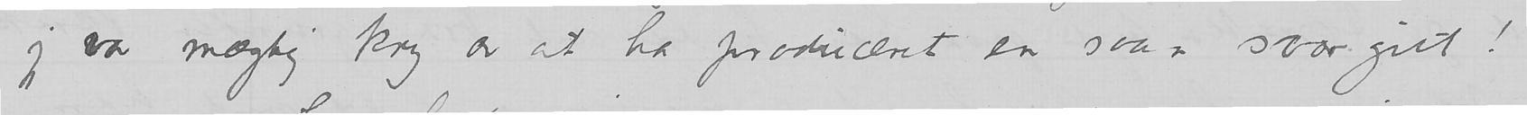jeg var mægtig kry over at ha produceret en saan svær gut!
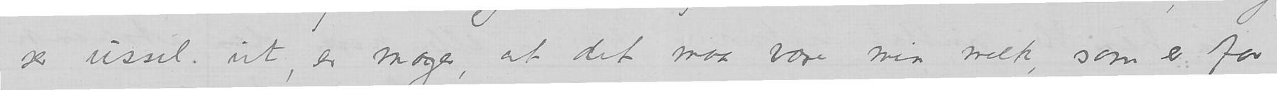ser ussel ut, er mager, at det maa væære min melk, som er for
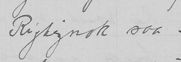Rigtignok saa
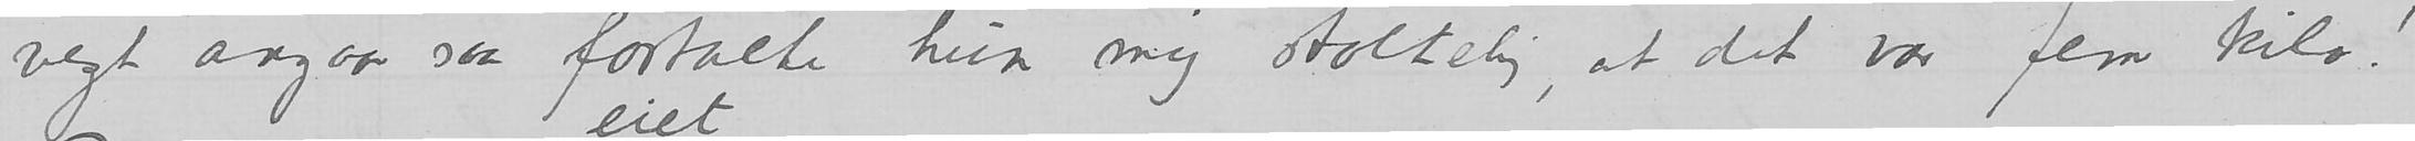vegt angaar saa fortalte hun mig stoltelig, at det var fem kilo!
